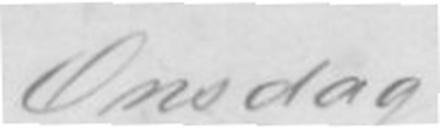Onsdag
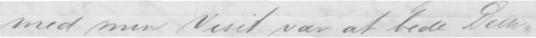med min Visit var at bede Dem
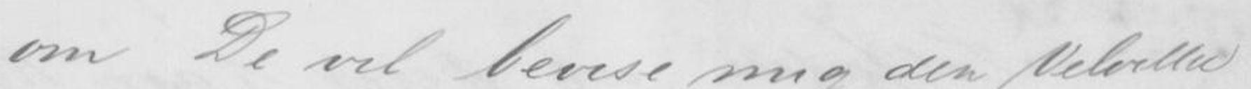om De vil bevise mig den Velvilhed
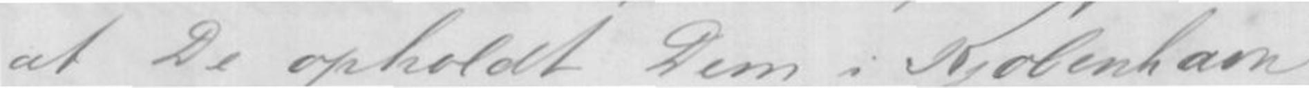at De opholdt Dem i Kjøbenhavn
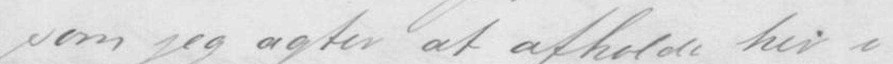som jeg agter at afholde her i
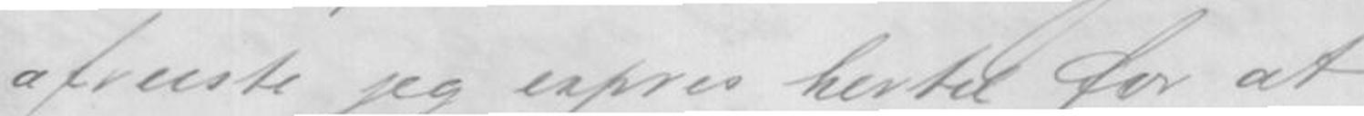afreiste jeg expres hertil for at
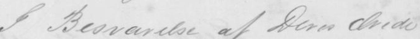I Besvarelse af Deres Ærede
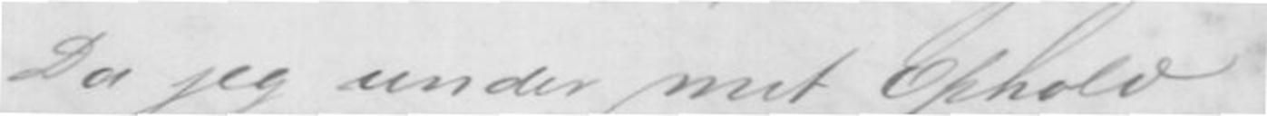Da jeg under mit Ophold
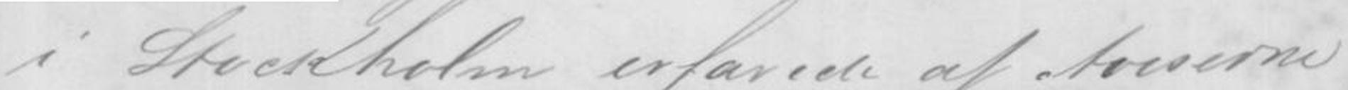i Stockholm erfarede af Aviserne
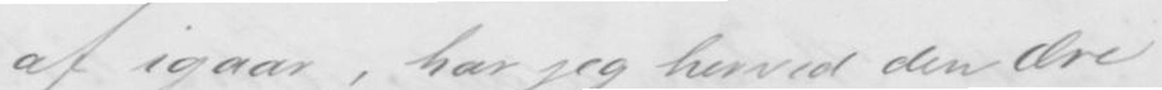af igaar, har jeg herved den Ære
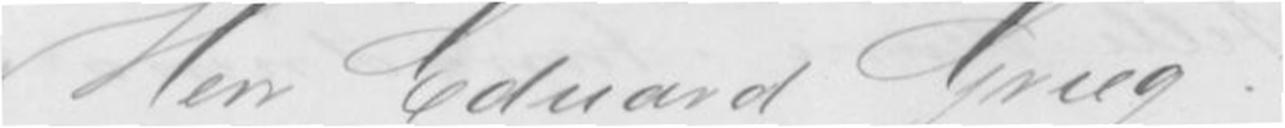Herr Edvard Grieg!
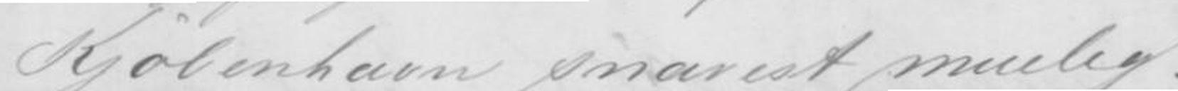Kjøbenhavn snarest mulig.
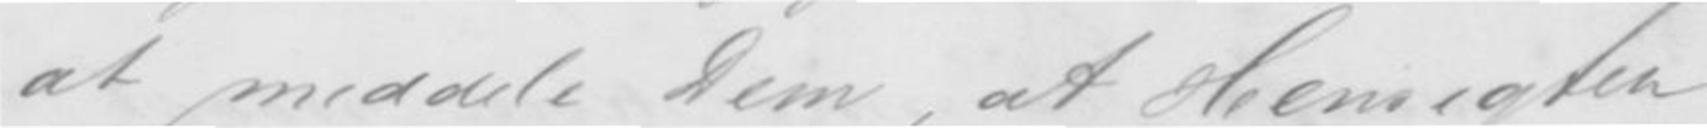at meddele Dem, at Hensigten
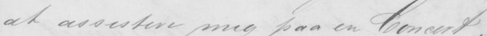at assistere mig paa en Concert,
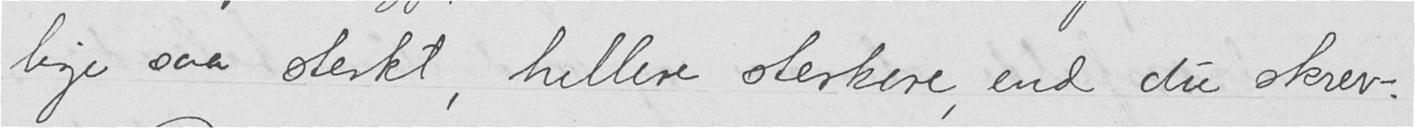lige saa sterkt, hellere sterkere, end du skrev.
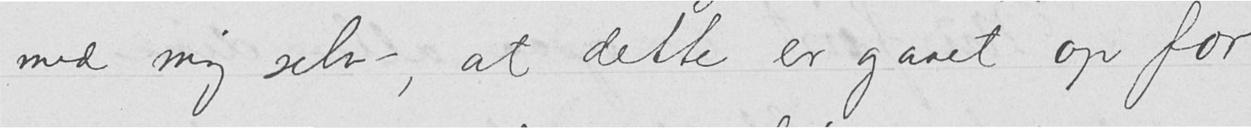med mig selv, at dette er gaaet op for
